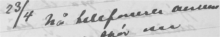23/4 Nå telefonerer avisene
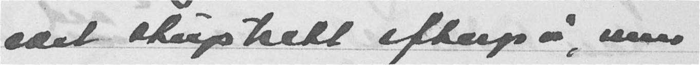vært stuptrett efterpa, men
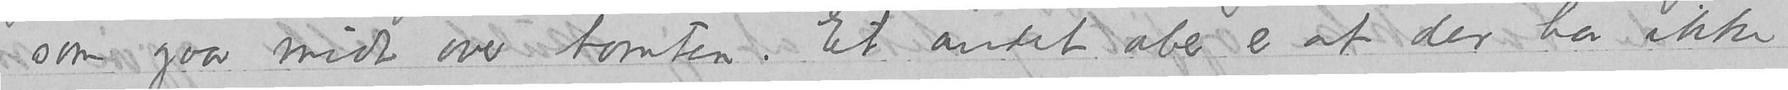som gaar midt over tomten. Et andet aber er at der har ikke
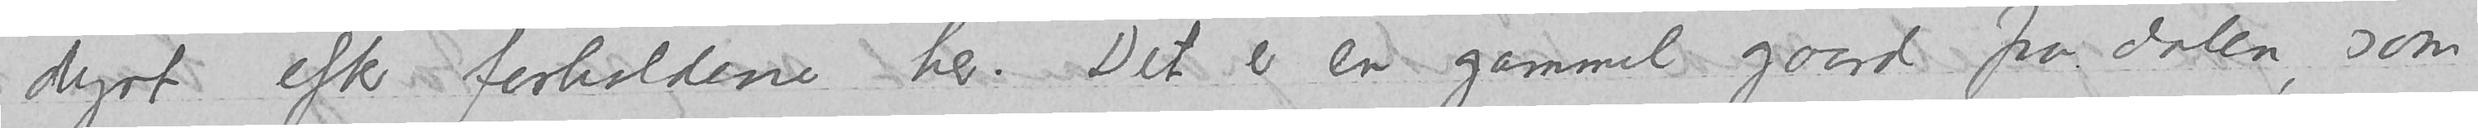dyrt efter forholdene her. Det er en gammel gaard fra dalen, som
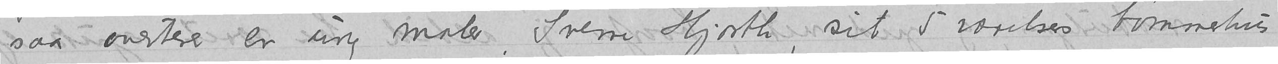saa averterer en ung maler, Sverre Hjorth, sit 5 værelses tømmerhus
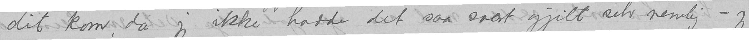dit kom, da jeg ikke hadde det saa svært gjilt selv nemlig – jeg
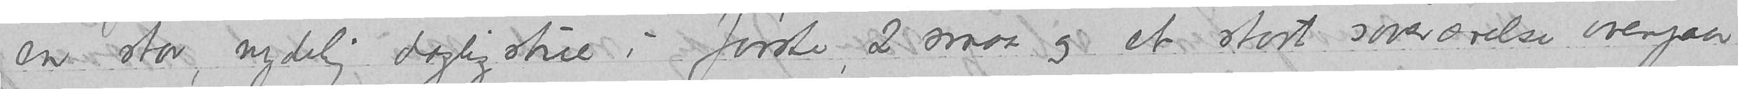en stor, nydelig, dagligstue i første, 2 smaa og et stort soveværelse ovenpaa.
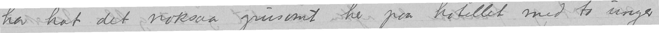har hat det noksaa grusomt her paa hotellet med to unger
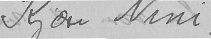Kjære Nini,
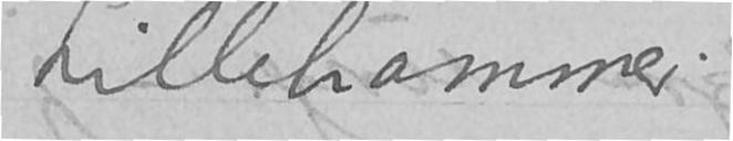Lillehammer.
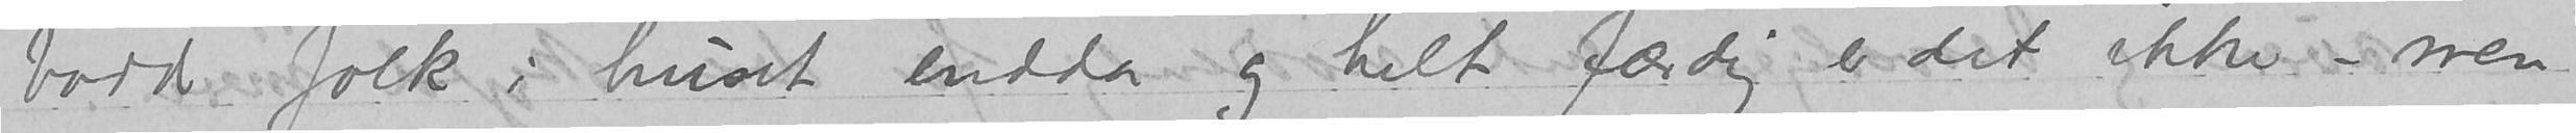bodd folk i huset endda og helt færdig er det ikke – men
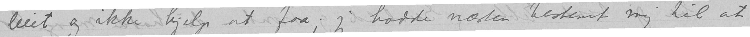leiet og ikke hjelp at faa; jeg hadde næsten bestemt mig til at
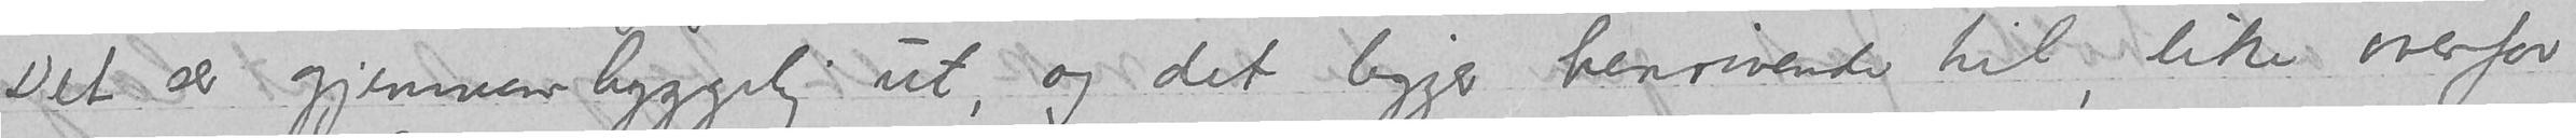Det ser gjennemhyggelig ut, og det ligger henrivende til, like overfor
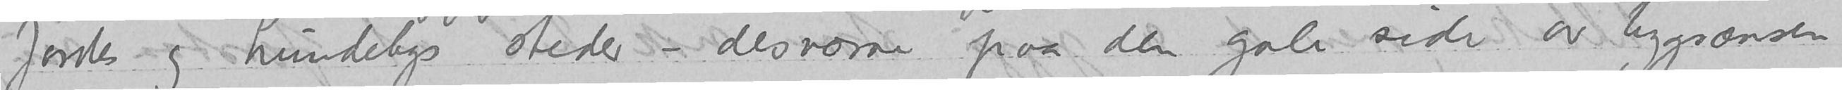Jordes og Lundebys steder – desværre paa den gale side av bygrænsen
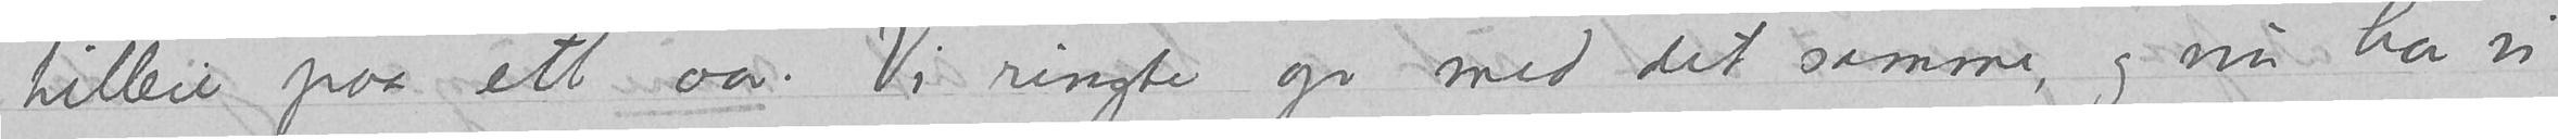tilleie paa ett aar. Vi ringte op med det samme, og nu har vi
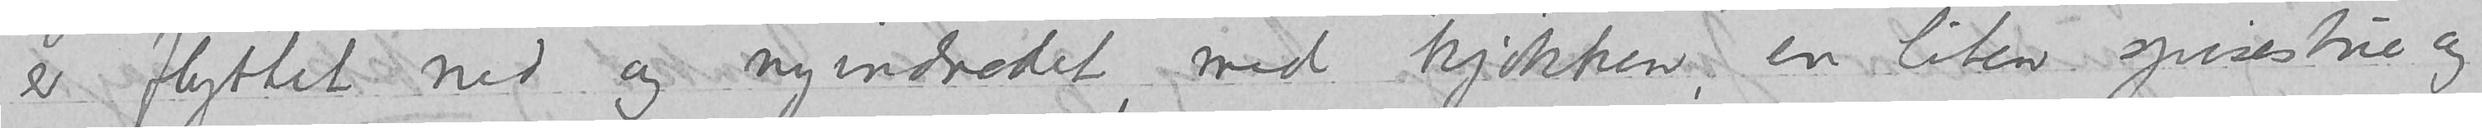er flyttet ned og nyindrædet, med kjøkken, en liten spisestue og
